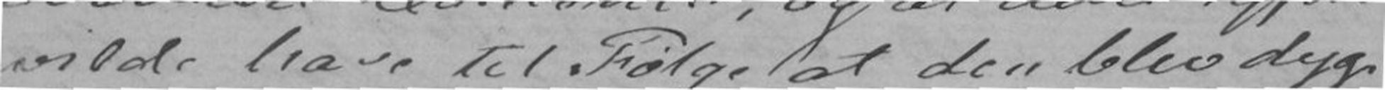vilde have til Følge at den blev dyg-
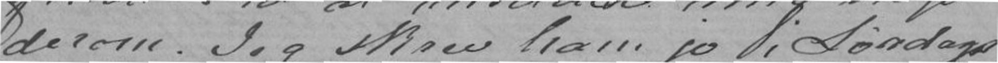derom. Jeg skrev ham jo i Lørdags
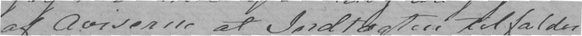af Aviserne at Indtægten tilfalder
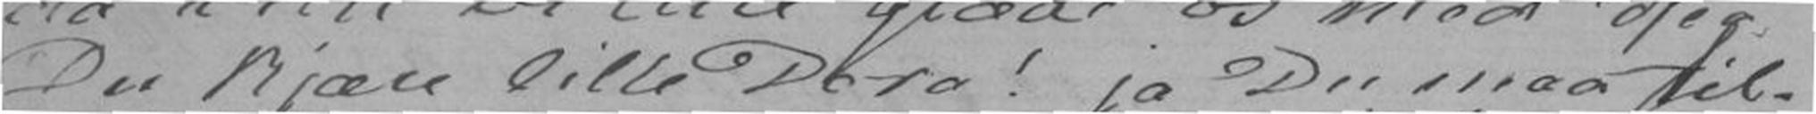Du kjære lille Dora! ja Du maa til-
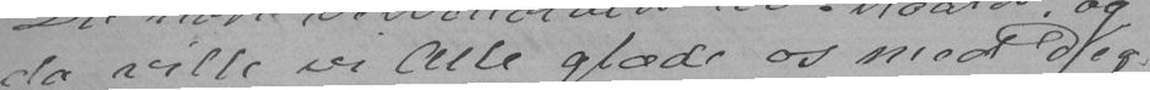da ville vi Alle glæde os med Deg
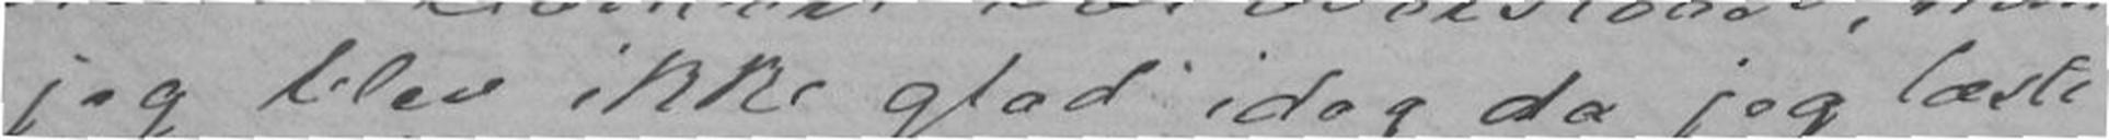jeg blev ikke glad idag da jeg læste
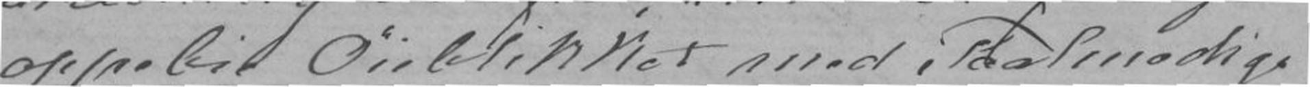oppebie Øieblikket med Taalmodig-
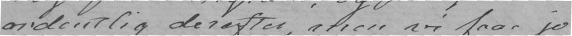ordentlig derefter, men vi faar jo
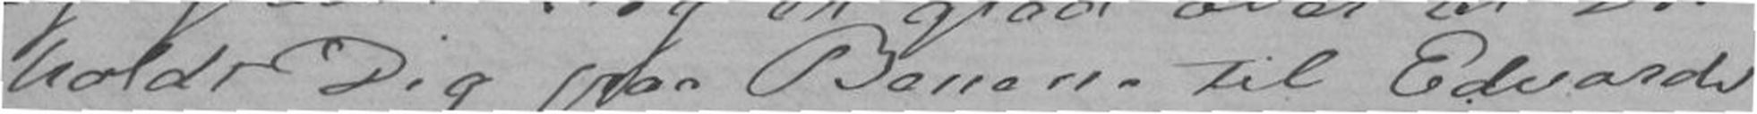holdt Dig paa Benene til Edvards
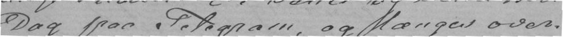Dag paa Telegram, og længes over-
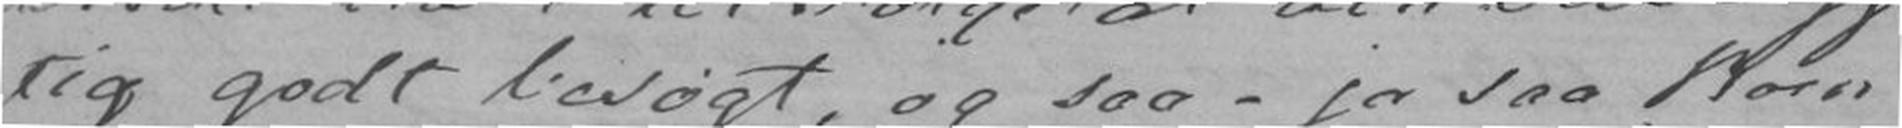tid godt besøgt, og saa - ja saa kom
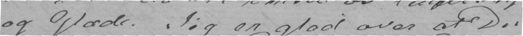og Glæde. Jeg er glad over at Du
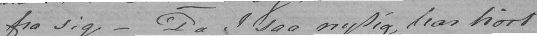fra sig - Da I saa nylig har hørt
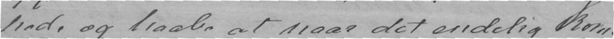hed, og haaber at naar det endelig kom-
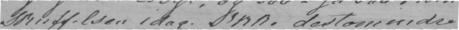skuffelsen idag. Ikke destomindre
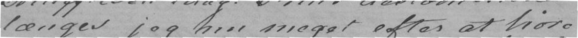længes jeg nu meget efter at høre
